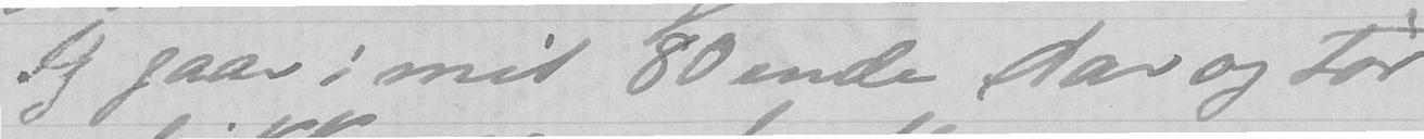Jeg gaar i mit 80ende Aar og tør
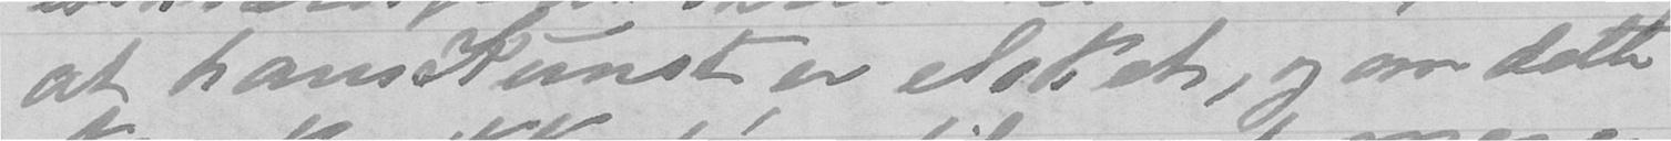at hans Kunst er elsket, og om dette
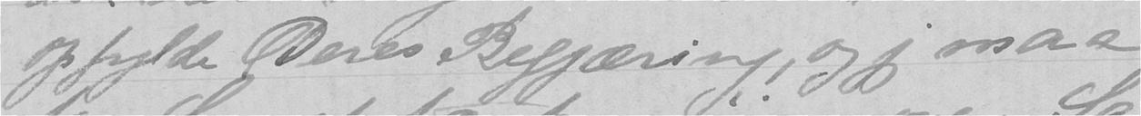opfylde Deres Begjæring, og jeg maa
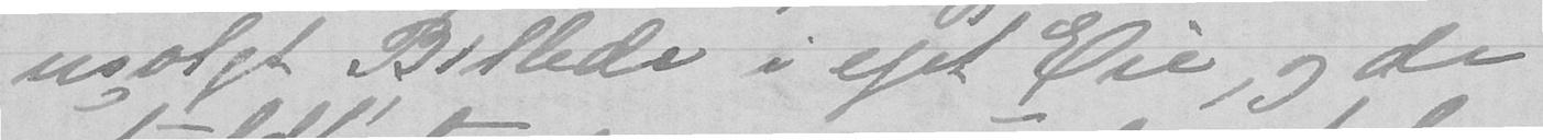usolgt Billede i eget Eie, og de
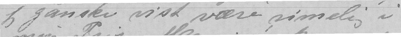jeg ganske vist være rimelig i
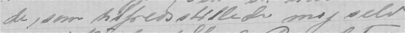de, som tilfredsstillede mig selv
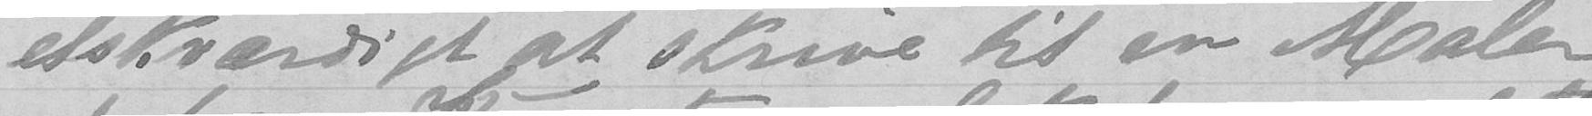elskværdigt at skrive til en Maler
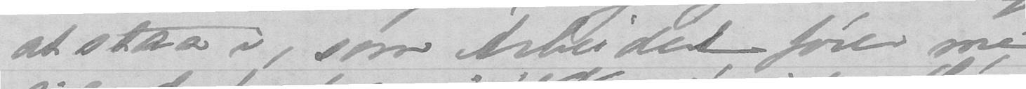at staa i, som Arbeidet fører med
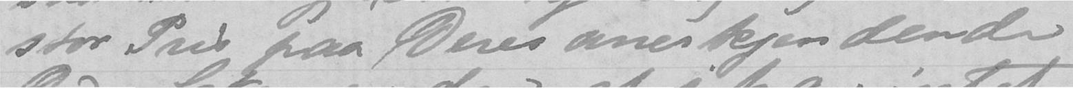stor Pris paa Deres anerkjendende
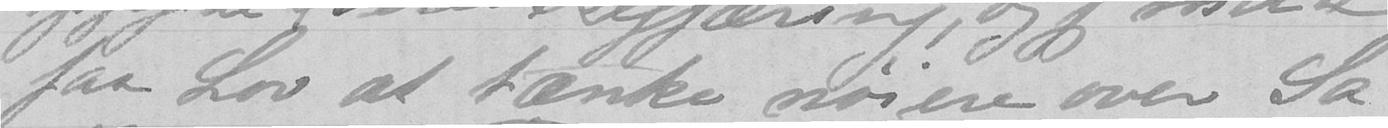faa Lov at tænke nøiere over Sa-
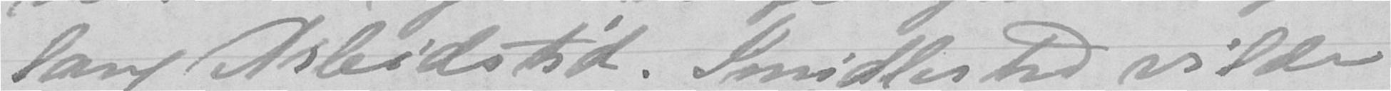lang Arbeidstid. Imidlertid vilde
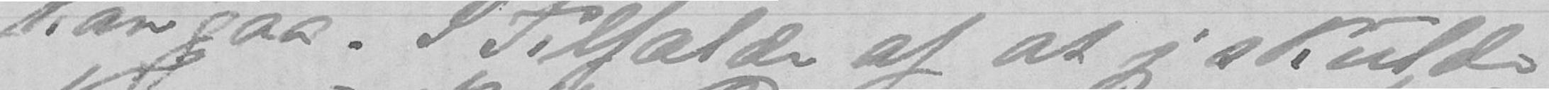kan gaa. I Tilfælde af at jeg skulde
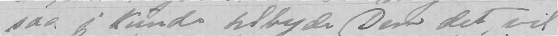saa jeg kunde tilbyde Dem det, vil
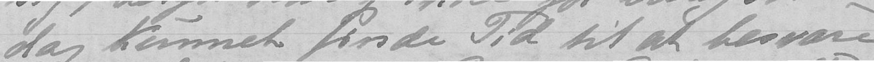dag kunnet finde Tid til at besvare
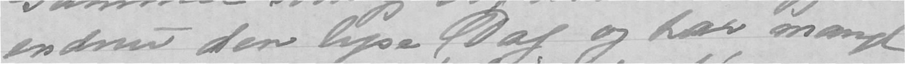endnu den lyse Dag og har mangt
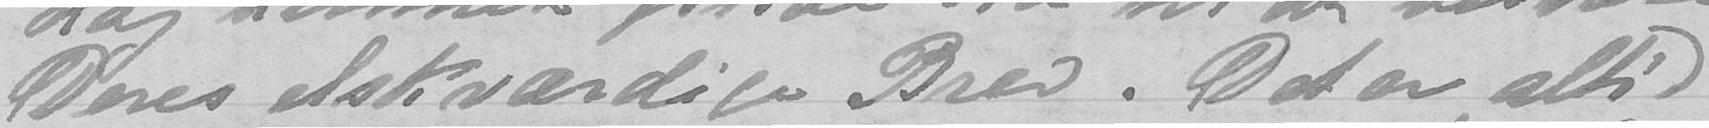Deres elskværdige Brev. Det er altid
